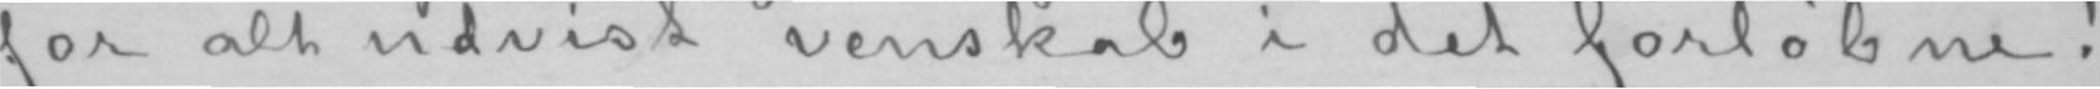for alt udvist venskab i det forløbne!
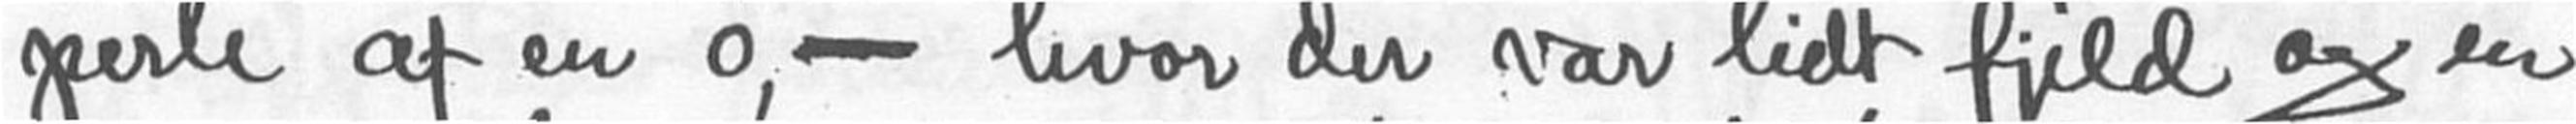perle af en ø, - hvor der var lidt fjeld og en
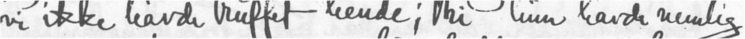vi ikke hadde truffet hende ; thi hun havde nemlig
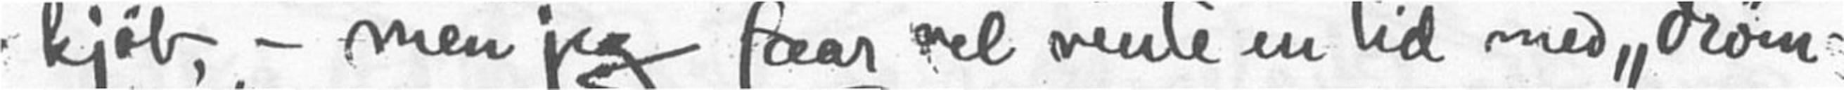kjøb,- men jeg faar vel vente en tid med "drøm-
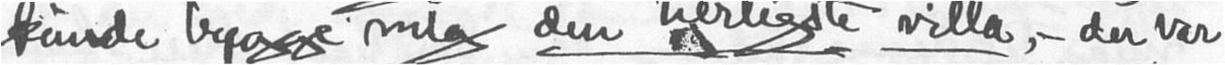kunde bygge mig den herligste villa,- der var
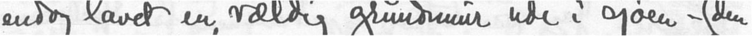endog lavet en vældig grundmuur ude i sjøen - (den
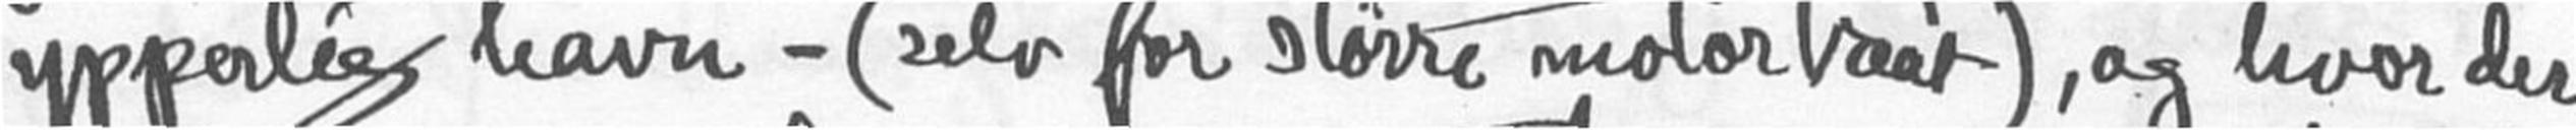ypperlig havn - (selv for større motorbaat), og hvor der
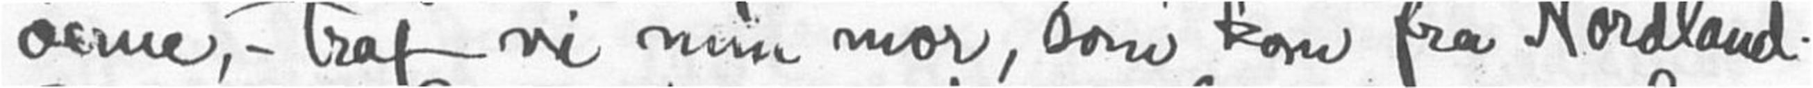øerne, - traf vi min mor, som kom fra Nordland.
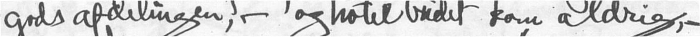godsafdelingen,- og hotelbudet kom aldrig, -
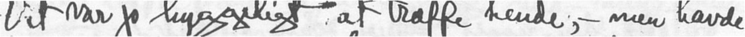Det var jo hyggeligt at træffe hende, - men havde
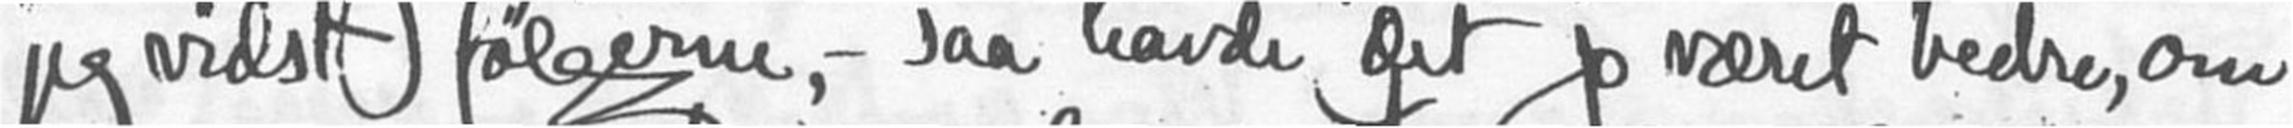jeg vidst følgerne, - saa havde det jo været bedre, om
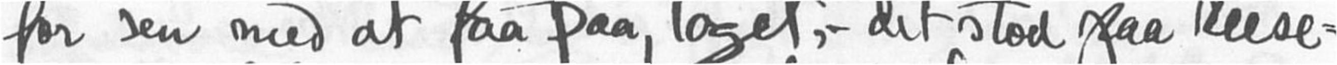for sen med at faa paa toget, - det stod paa reise-
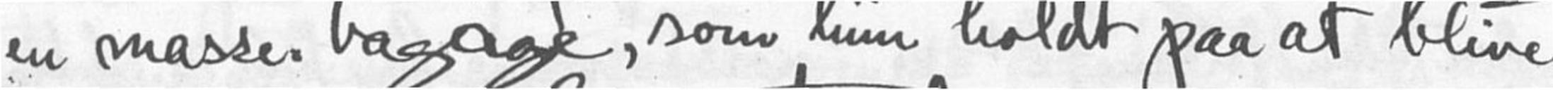en masse bagage, som hun holdt paa at blive
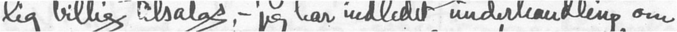lig billig tilsalgs, - jeg har indledet underhandling om
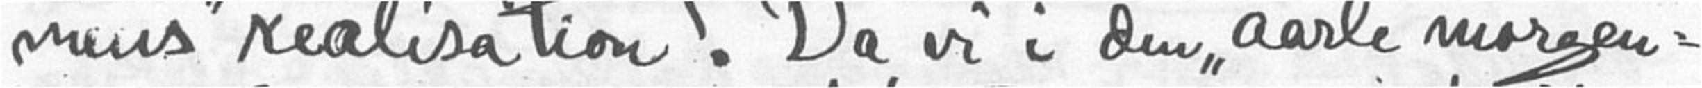mens" realisation. Da vi i den "aarle" morgen-
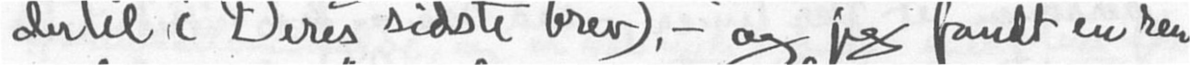dertil i Deres sidste brev), - og jeg fandt en ren
